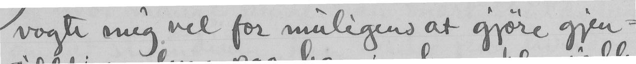vogte mig vel for muligens at gjøre gjen-
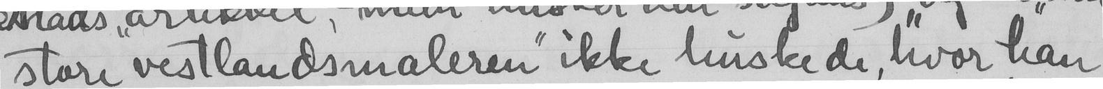store vestlandsmaleren" ikke huskede, hvor han
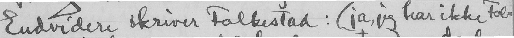Endvidere skriver Folkestad: (ja, jeg har ikke Fol-
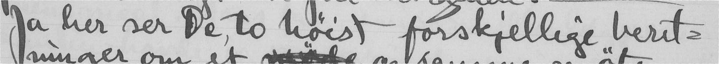Ja her ser De, to høist forskjellige beret-
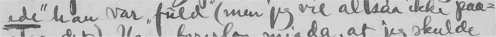ede" han var "fuld" (men jeg vil altsaa ikke paa-
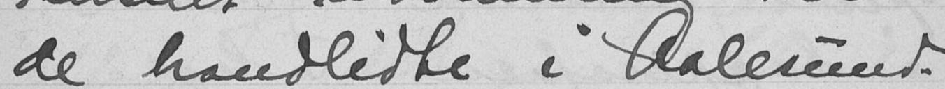de brandlidte i Aalesund.
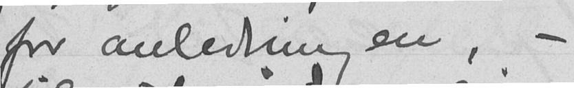for anledningen, -
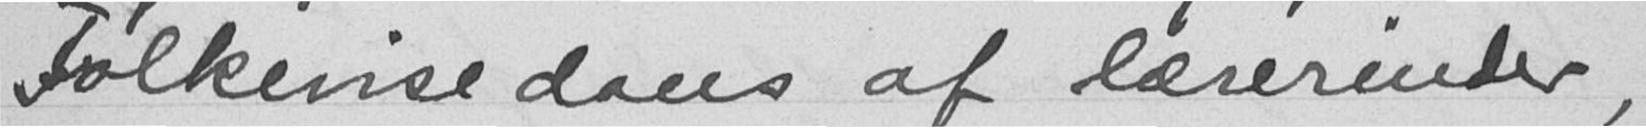Folkevisedans af lærerinder,
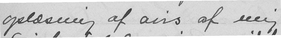oplæsning af avis af mig
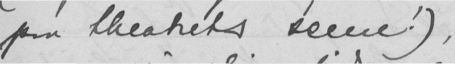paa theatrets scene!),
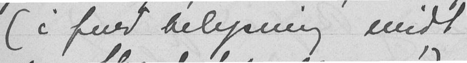(i fuld belysning midt
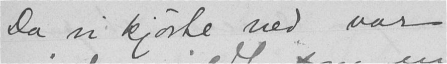Da vi kjørte ned var
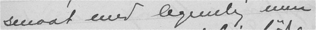smaat med legemlig men
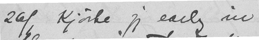26/1 Kjørte jeg early in
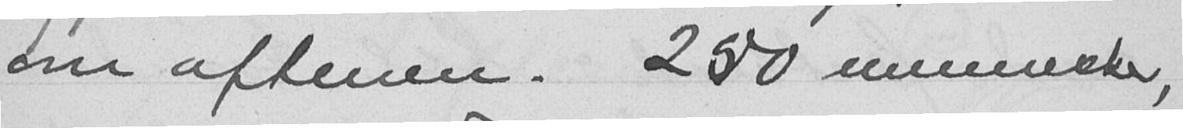om aftenen. 250 mennesker,
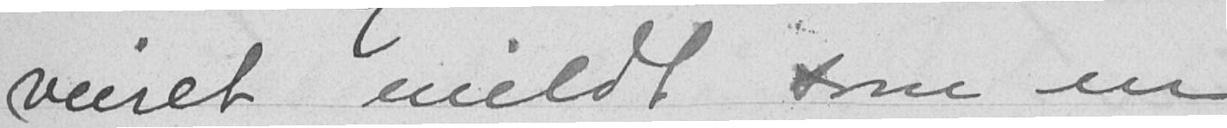veiret mildt som en
